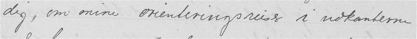dig, om mine orienteringsreiser i udkanterne
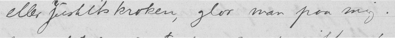eller Justitskroken, glor man paa mig.
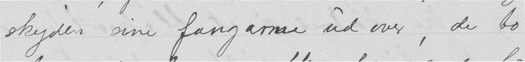skyder sine fangarme ud over, de to
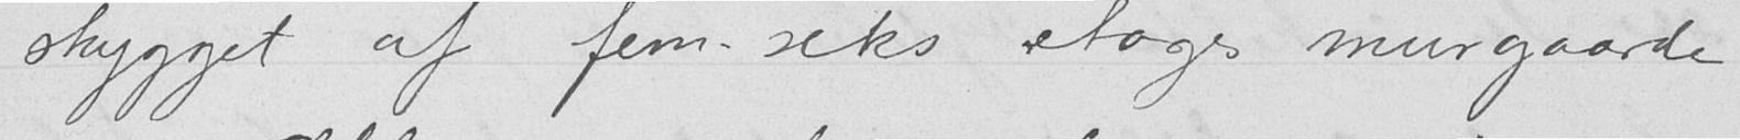skygget af fem-seks etages murgaarde,
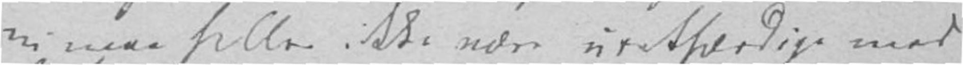vi maa heller ikke være uretfærdige mod
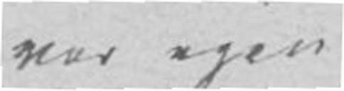vor egen
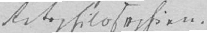Retsphilosophien.
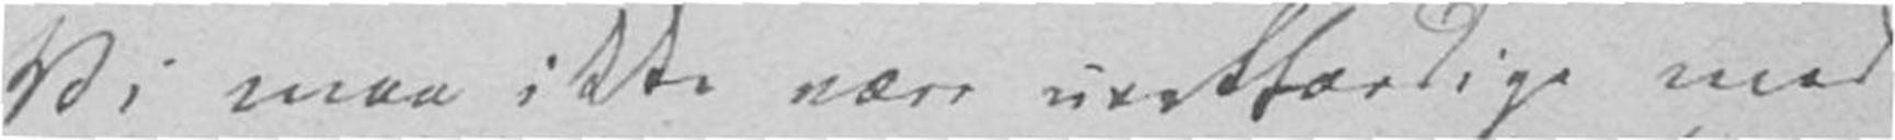Vi maa ikke være uretfærdige mod
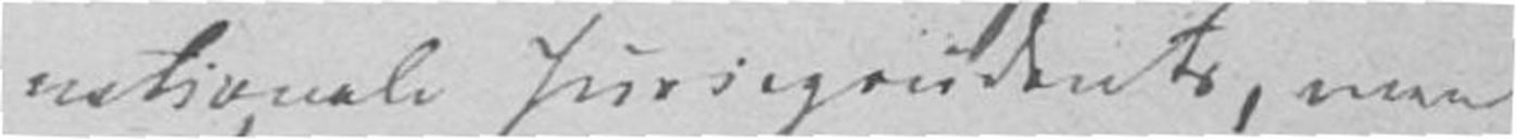nationale Jurisprudents, men
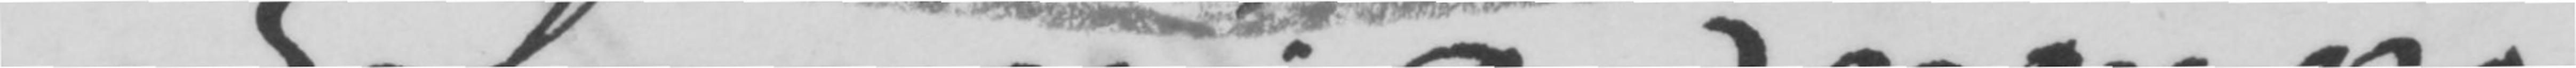Konsul D., som jeg i høst traf hos Dem,
